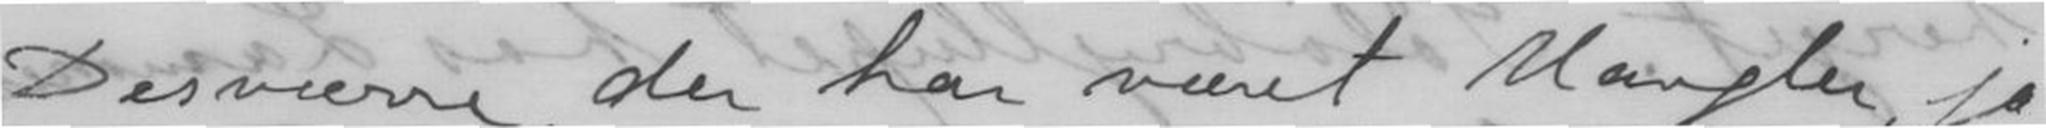Desværre, der har været Mangler, jo
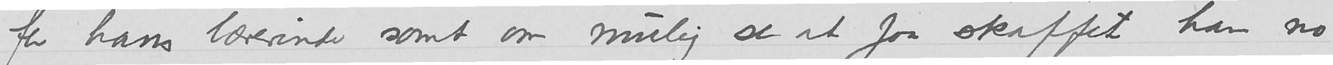for hans lærerinde samt om mulig se at faa skaffet ham no
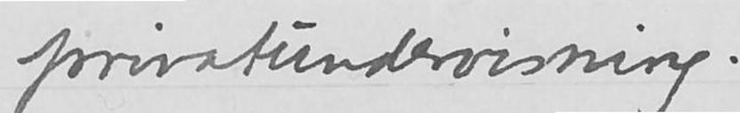privatundervisning.
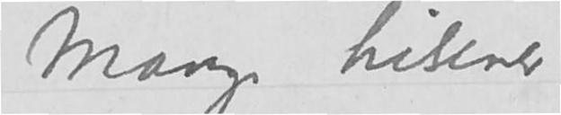Mange hilsener
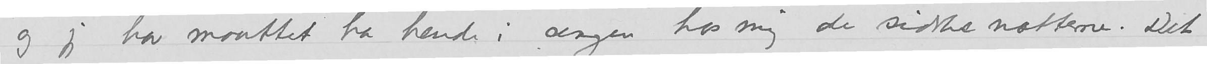og jeg har maattet ha hende i sengen hos mig de sidste nætterne. Det
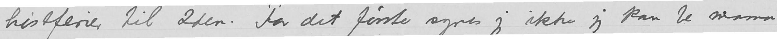høstferie til 2den. For det første synes jeg ikke jeg kan be mama
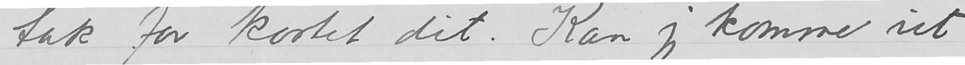tak for kortet dit. Kan jeg komme ut
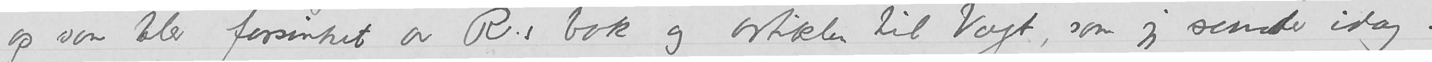op som blev forsinket av R.s bok og artiklen til Vogt, som jeg sender idag.
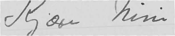Kjære Nini,
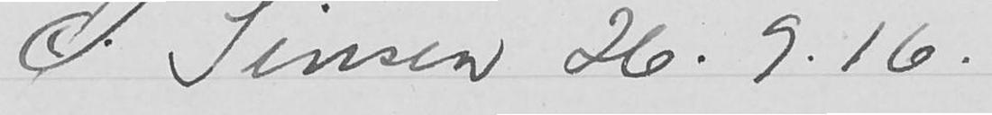Ø. Sinsen 26.9.16.
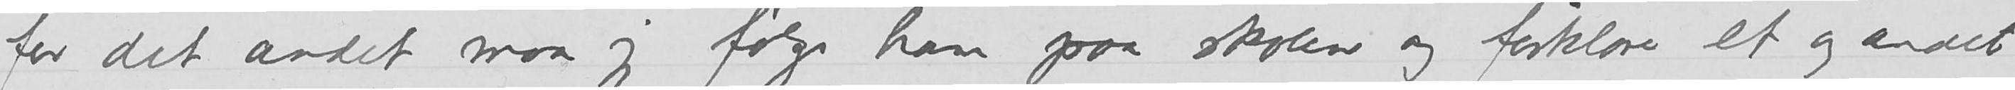for det andet maa jeg følge ham paa skolen og forklare et og andet
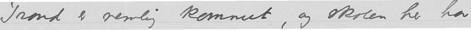Trond er nemlig kommet, og skolen her har
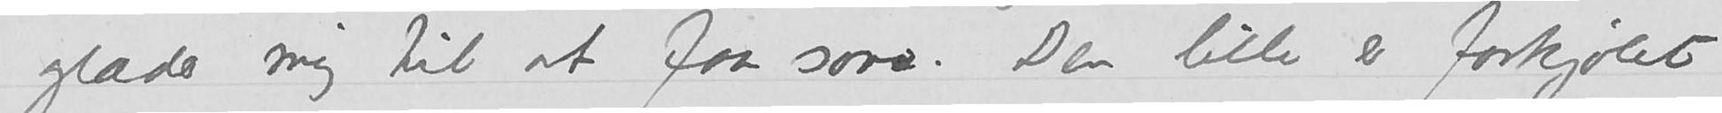Jeg glæder mig til at faa sove. Den lille er forkjølet
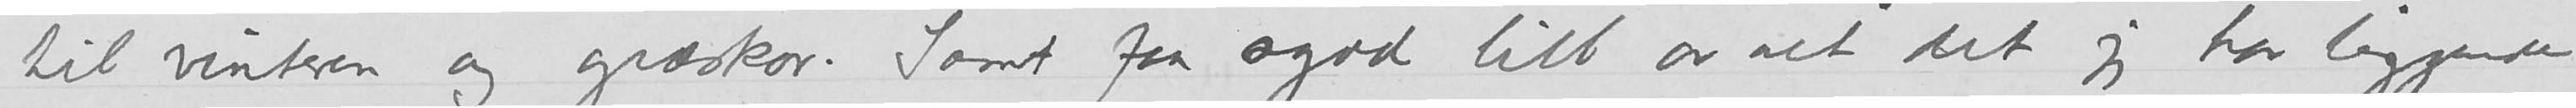til vinteren og græskar. Samt faa sydd litt av alt det jeg har liggende
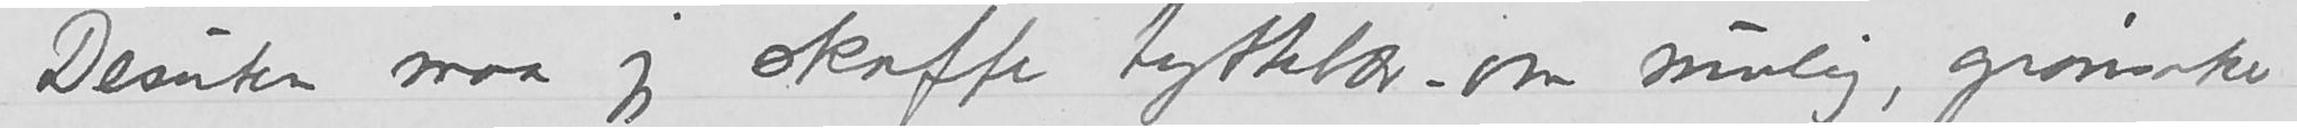Dessuten maa jeg skaffe tyttebær – om mulig, grønsaker
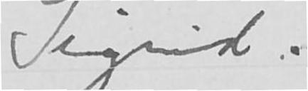Sigrid.
